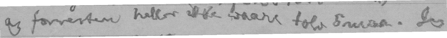og forresten heller ikke vaare tolv Smaa. Jeg
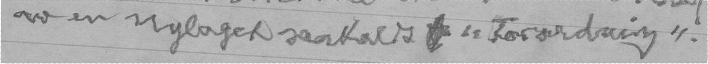av en nylaget saakaldt  "Forordning".
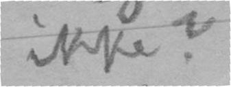ikke?
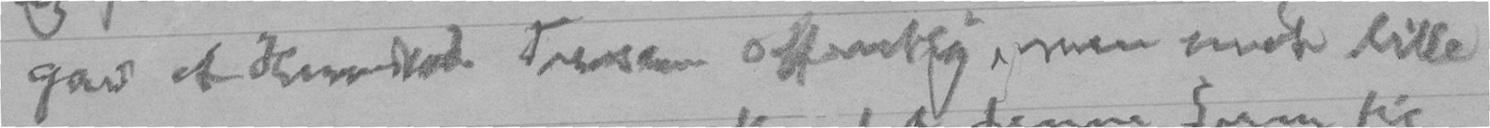gav et Hundred Tusen offentlig, men mit lille
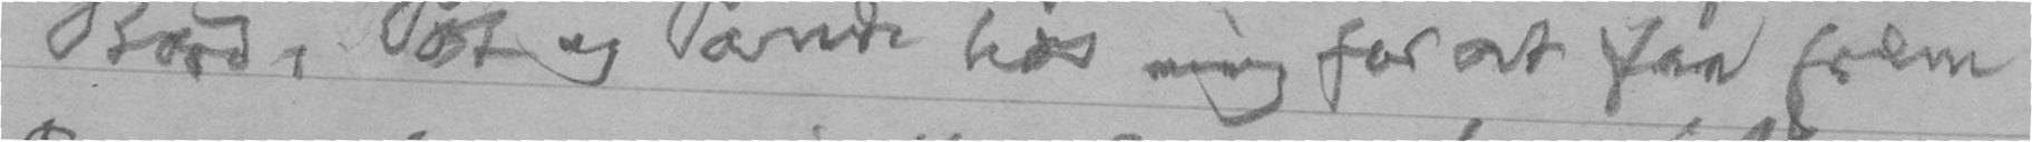Bord, Pot og Pande hos mig for at faa frem
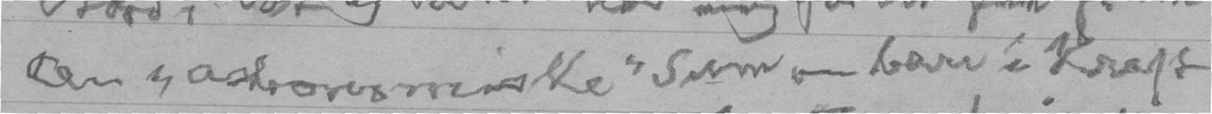den "astronomiske" Sum - bare i Kraft
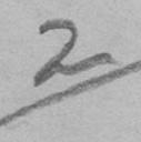2
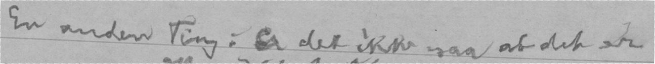En anden Ting: Er det ikke saa at det er
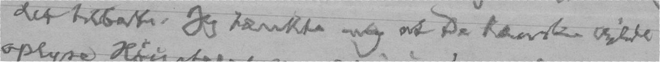det tilbake Jeg tænkte mig at De kanske vilde
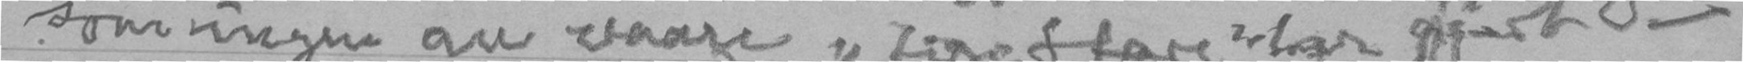som ingen av vaare "fire Store" har gjort -
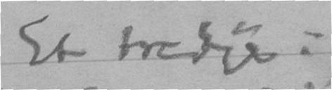Et tredie:
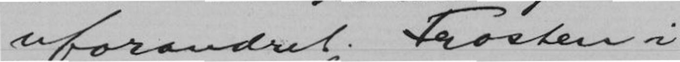uforandret. Frosten i
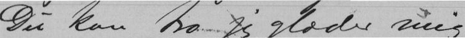Du kan tro jeg glæder mig
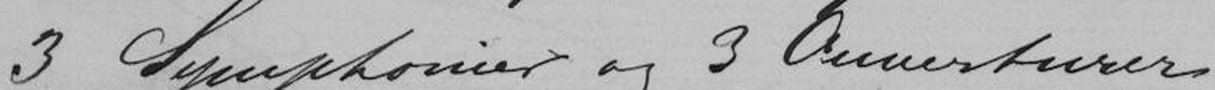3 Symphonier og 3 Ouverturer
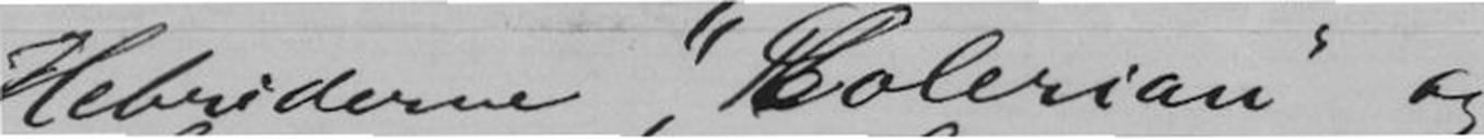Hebriderne, "Colerian" og
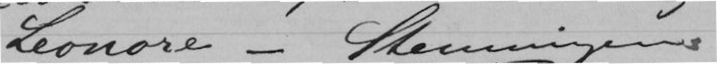Leonore - Stemningen
